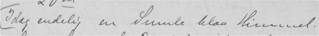Idag endelig en Smule blaa Himmel.
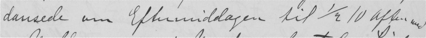dansede om Eftermiddagen til 1/2 10 Aften med
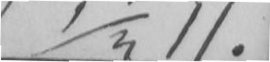1/2 11.
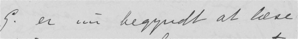G. er nu begyndt at læse
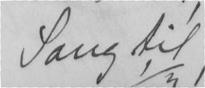Sang til
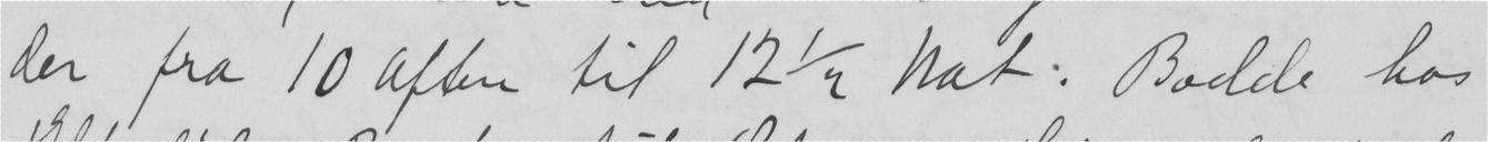der fra 10 Aften til 12½ Nat. Bodde hos
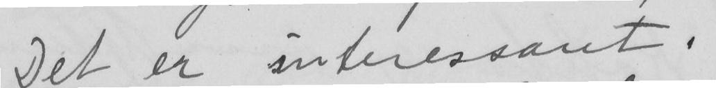Det er interessant.
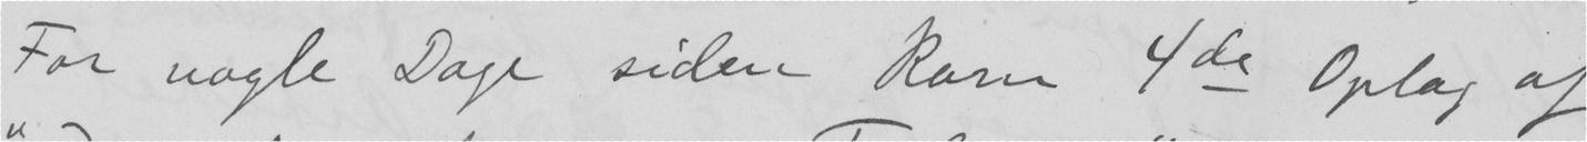For nogle Dage siden kom 4de Oplag af
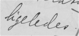ligeledes.
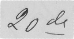20de
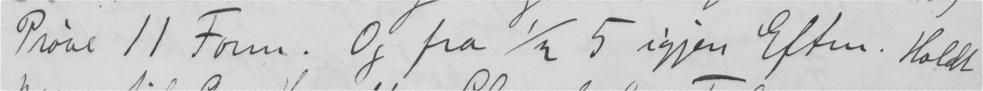Prøve 11 Form. Og fra 1/2 5 igjen Eften. Holdt
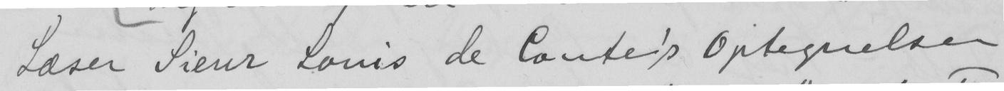Læser Sieur Louis de Conte's Optegnelser
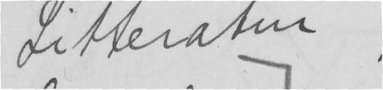Litterater"
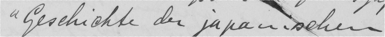"Geschickte der japanischen
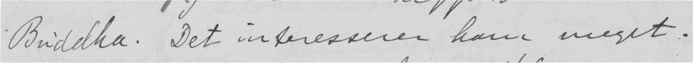Buddha. Det interesserer ham meget.
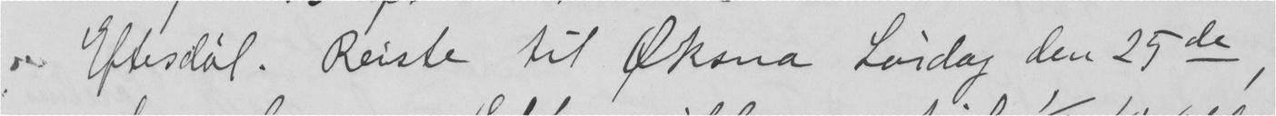Eftesdøl. Reiste til Øksna Lørdag den 25de,
